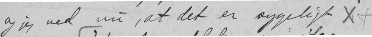og jeg ved nu, at det er sygeligt. x
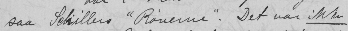saa Schillers "Røverne". Det var ikke
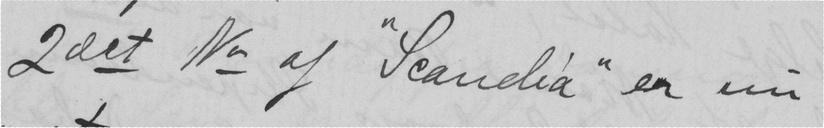2det No af "Scandia" er nu
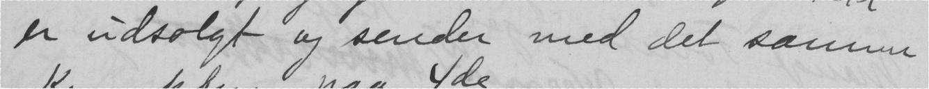er udsolgt og sender med det samme
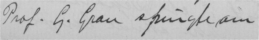Prof. G. Gran spurgte om
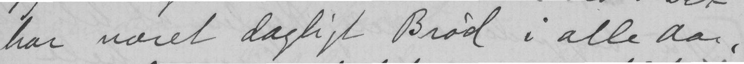har været dagligt Brød i alle aar,
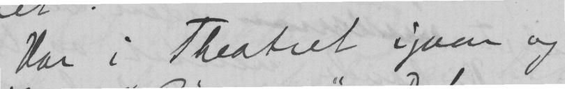Var i Theatret igaar og
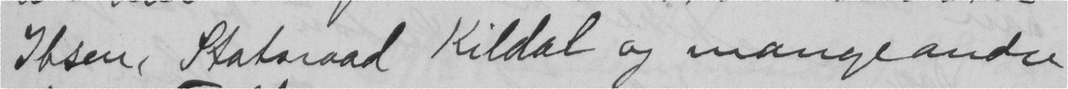Ibsen, Statsraad Kildal og mange andre
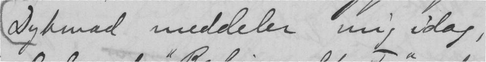Dybwad meddeler mig idag,
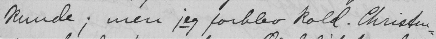kunde; men jeg forblev kold. Christen-
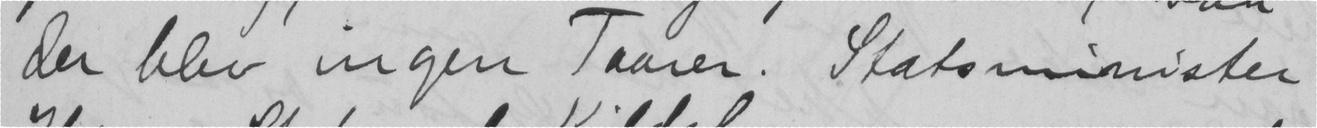der blev ingen Taarer. Statsminister
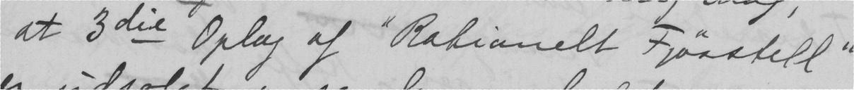at 3die Oplag af "Rationelt Fjøsstell"
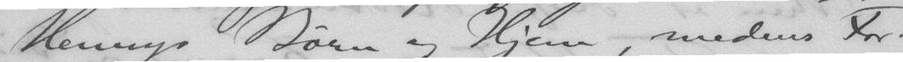Hennys Børn og Hjem, medens For-
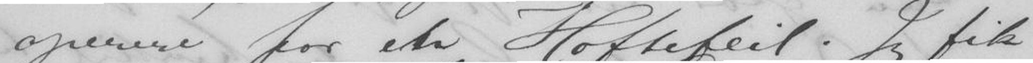operere for en Hoftefeil. Jeg fik
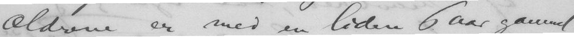ældrene er med en liden 6 Aar gammel
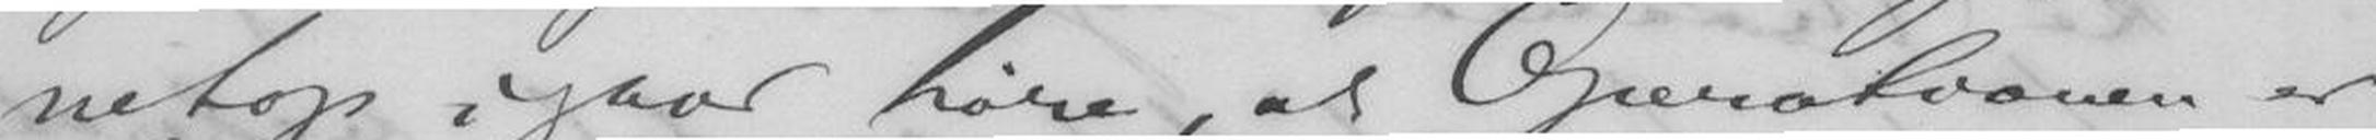netop igaar høre, at Operationen er
